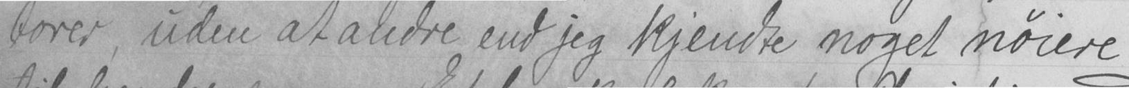torer, uden at andre end jeg kjendte noget nøiere
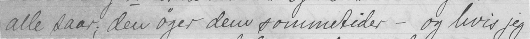alle saar; den øger dem sommetider - og hvis jeg
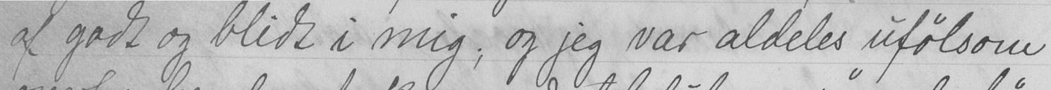af godt og blidt i mig; og jeg var aldeles ufølsom
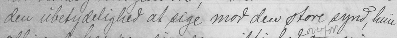den ubetydelighed at sige mod den store synd, hun
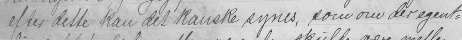efter dette kan det kanske synes, som om der egent-
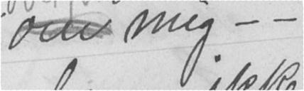om mig - -
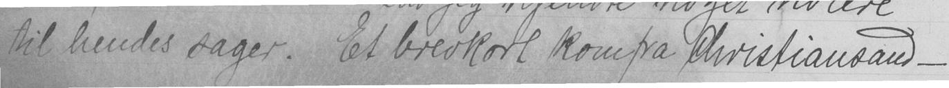til hendes sager. Et brevkort kom fra Christiansand -
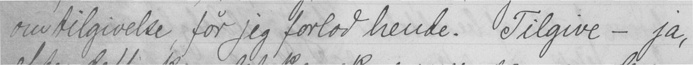om tilgivelse, før jeg forlod hende. Tilgive - ja,
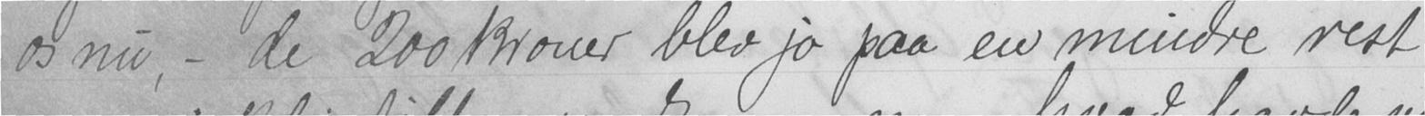os nu, - de 200 kroner blev jo paa en mindre rest
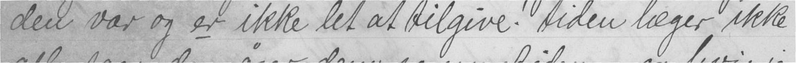den var og er ikke let at tilgive! tiden læger ikke
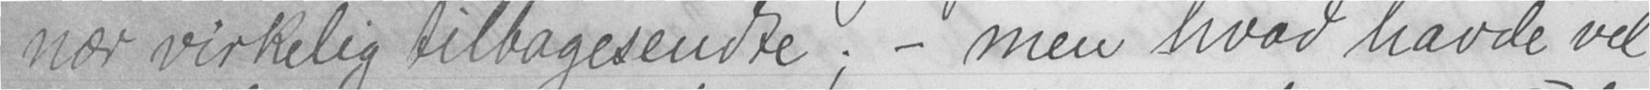nær virkelig tilbagesendte; - men hvad havde vel
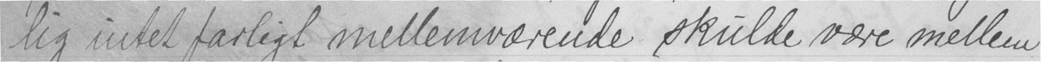lig intet farligt mellemværende skulde være mellem
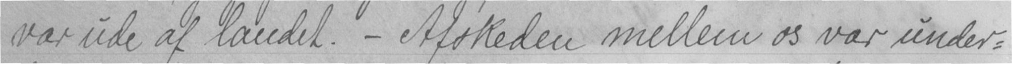var ude af landet. - Afskeden mellem os var under-
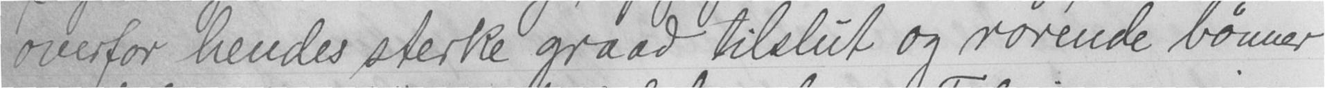overfor hendes sterke graad tilslut og rørende bønner
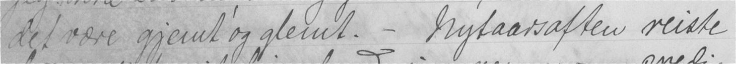det være gjemt og glemt. - Nytaarsaften reiste
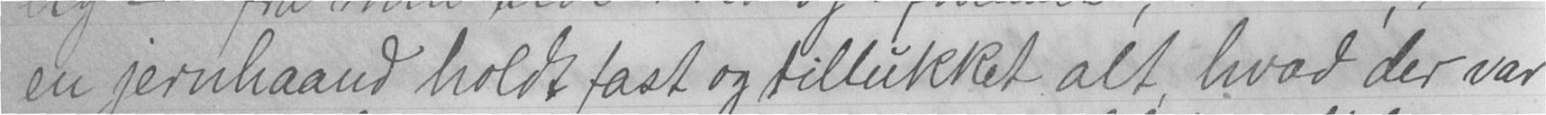en jernhaand holdt fast og tillukket alt, hvad der var
